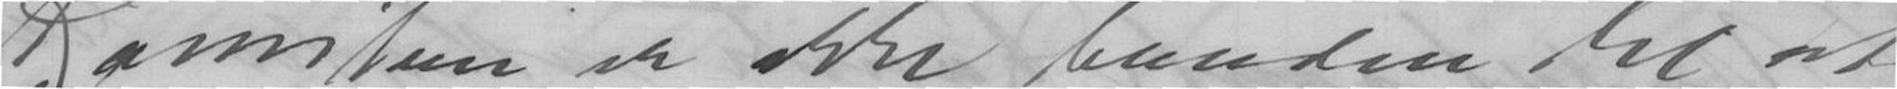Komiteen er ikke bunden til at
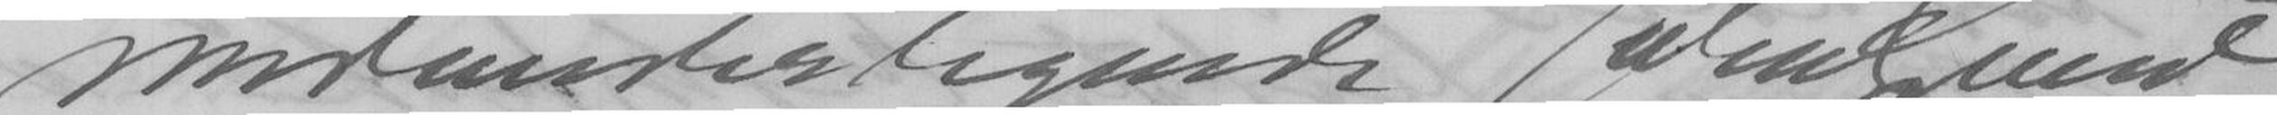medundertegnede John Lund
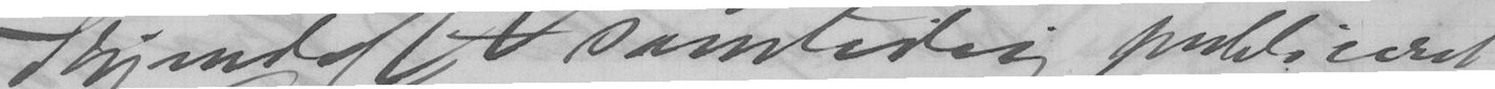Kjændrelse samtidig publiceret.
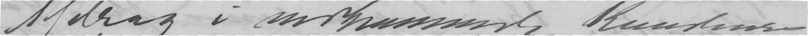Afdrag i vedkommende Kunstner
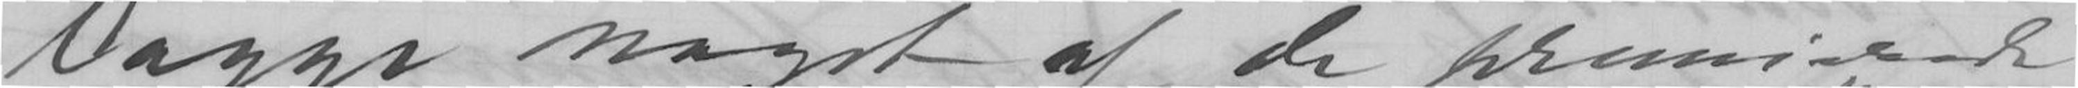lægge noget af de premierede
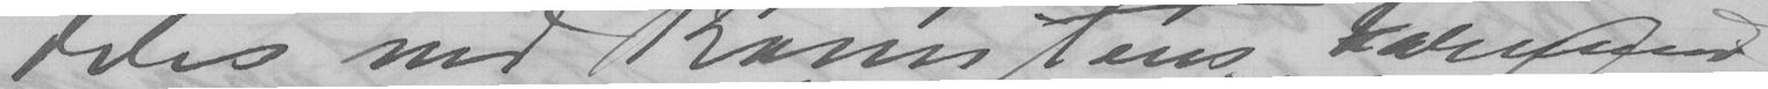deles ved Komiteens Formand
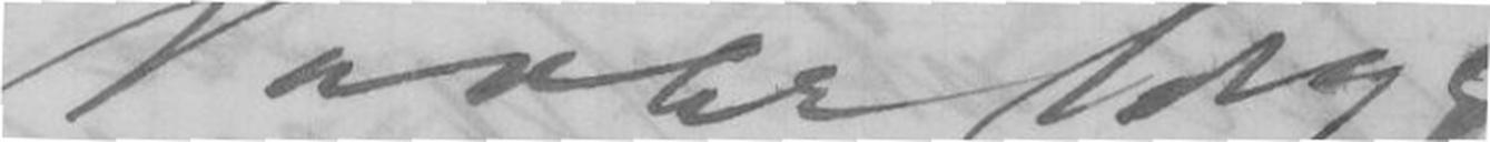Novbr 1895
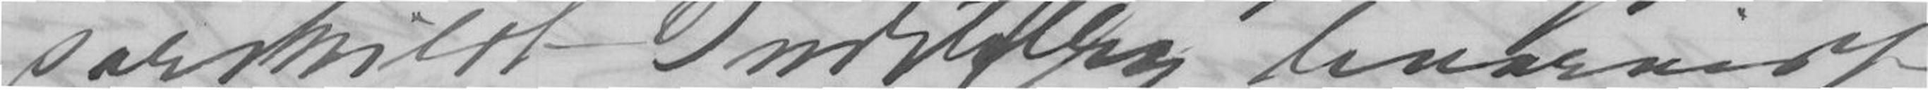særskilt Indstilling hvorvidt
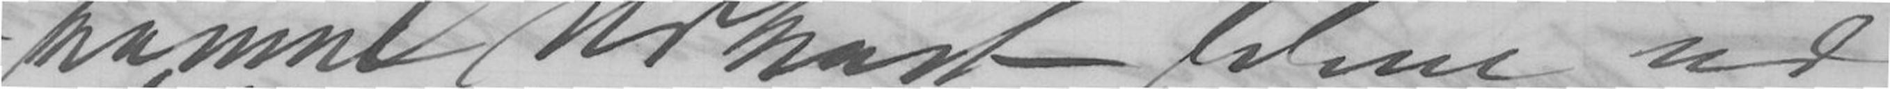komme Udkast blive ud-
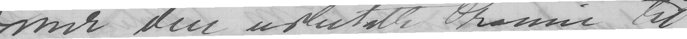mer den udbetalte Præmie til
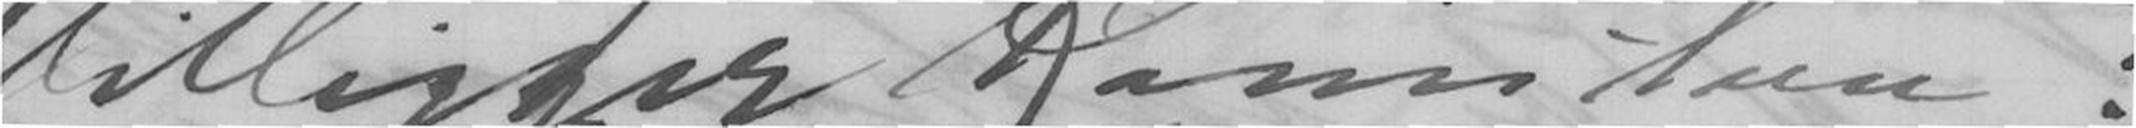tilligger Komiteen.
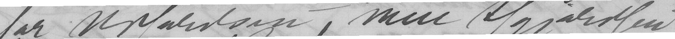for Udføreelsen, men Afgjørelsen
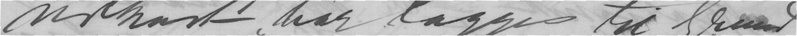udkast her lægges til Grund
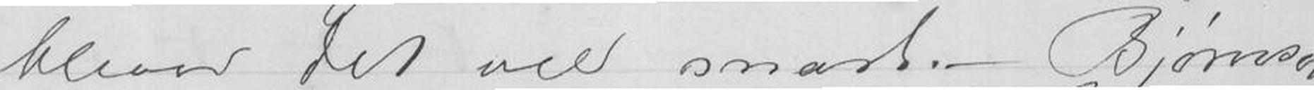bliver det vel snart. - Bjørnson
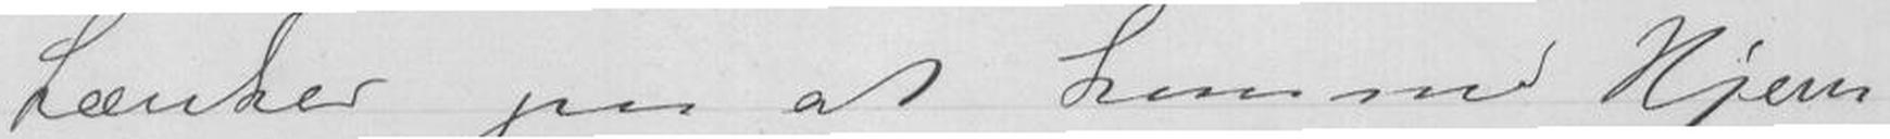tænker paa at komme Hjem
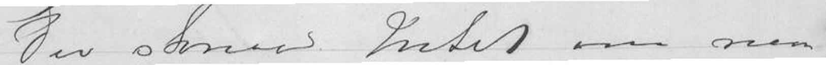Du skriver Intet om naar
